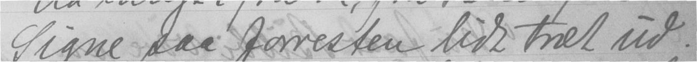Signe saa forresten lidt træt ud.
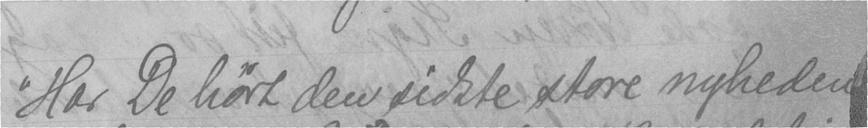"Har De hørt den sidste store nyheden
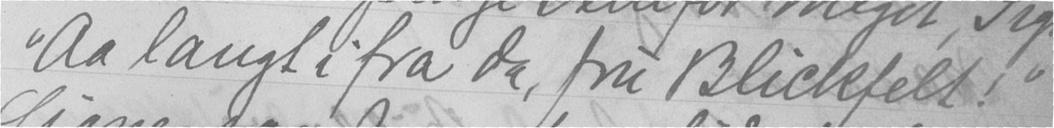"Aa langt i fra da, fru Blickfelt!"
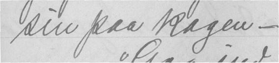sin paa kagen -
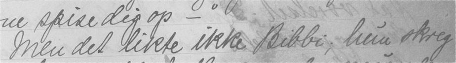Men det likte ikke Bibbi; hun skreg
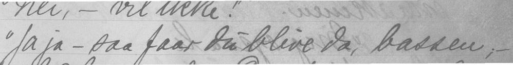"Ja, ja - saa faar du blive da, bassen; -
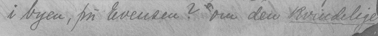i byen, fru Evensen? om den kvindelige
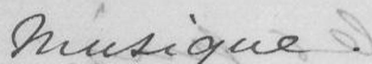Musique.
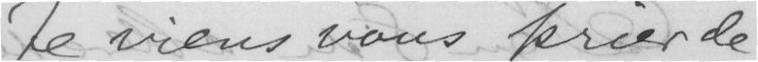Je viens vaus Prier de
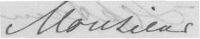Mousieur
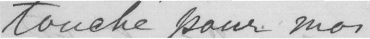touchè pour moi
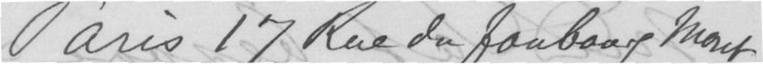Paris 17 Rue du foufaarg Marnt
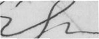E Gr.
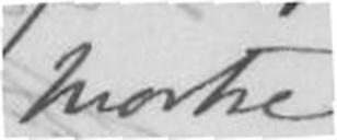Morte
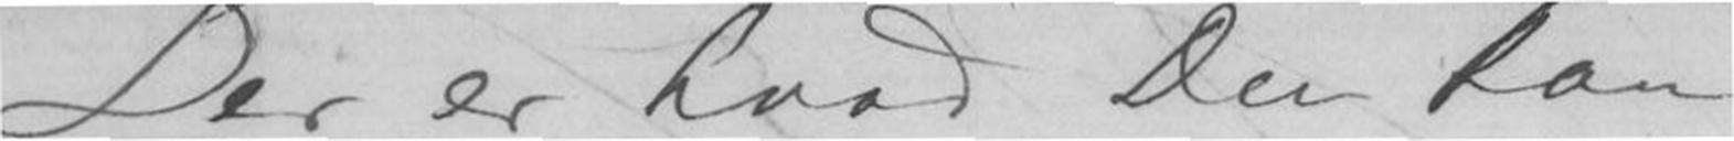Der er hvad Du kan
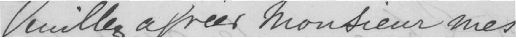Venillez afréer Monsieur mes
